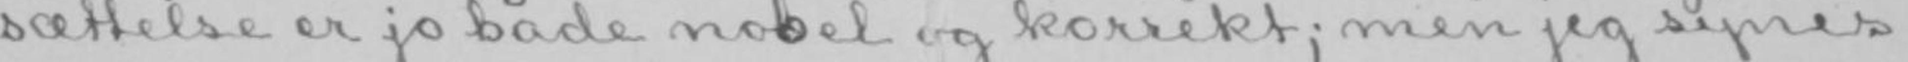sættelse er jo både nolbel og korrekt; men jeg synes
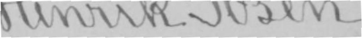Henrik Ibsen.
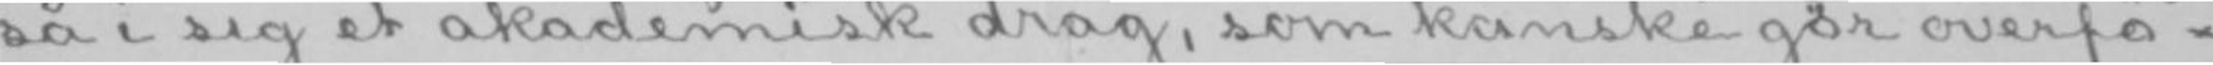så i sig et akademisk drag, som kanské gør overfø-
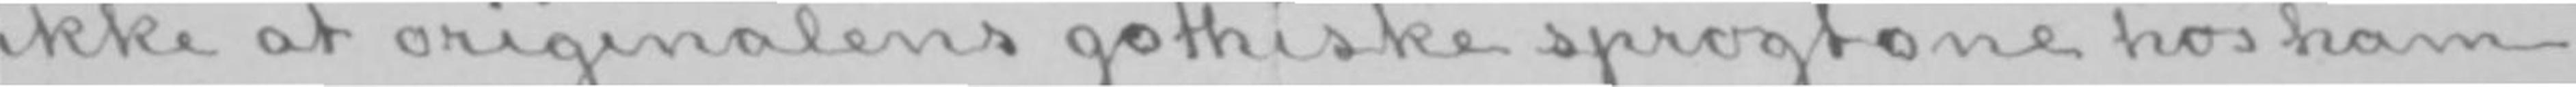ikke at originalens gothiske sprogtone hos ham
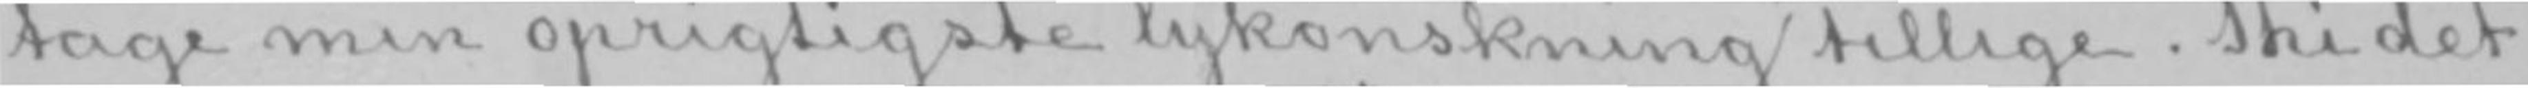tage min oprigtigste lykønskning tillige. Thi det
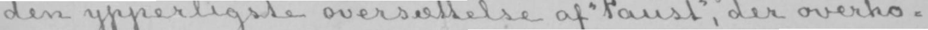den ypperligste oversættelse af «Faust», der overho-
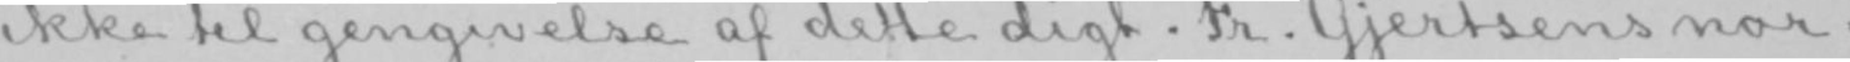ikke til gengivelse af dette digt. Fr. Gjertsens nor-
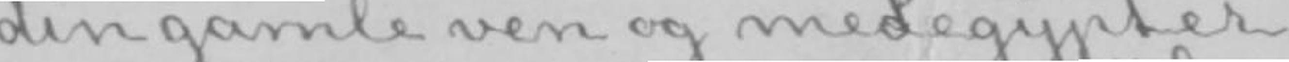din gamle ven og medegypter
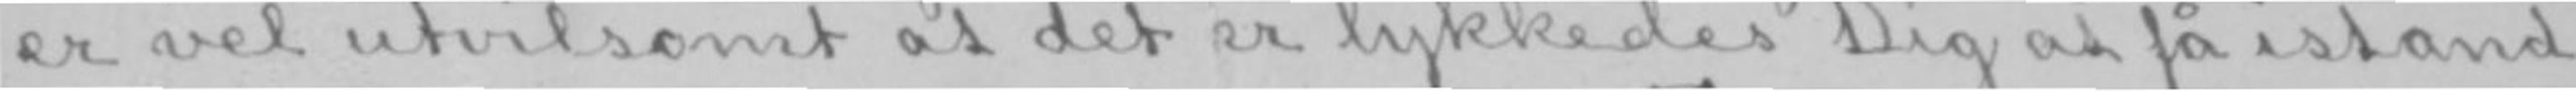er vel utvilsomt at det er lykkedes Dig at få istand
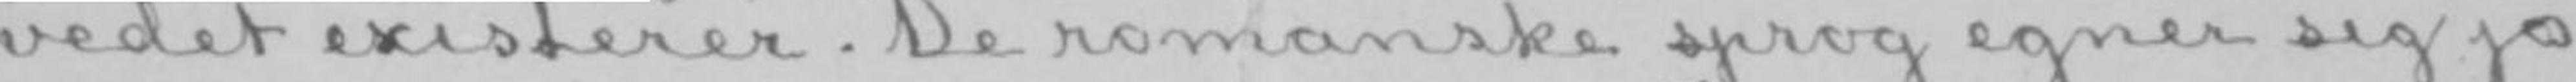vedet existerer. De romanske sprog egner sig jo
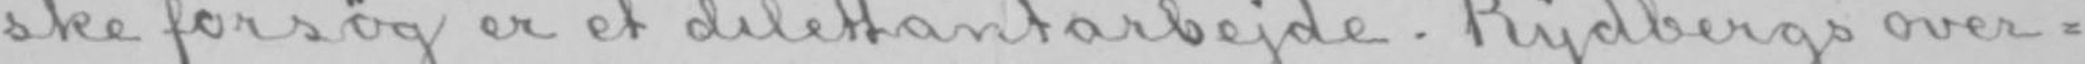ske forsøg er et dilettantarbejde. Rydbergs over-
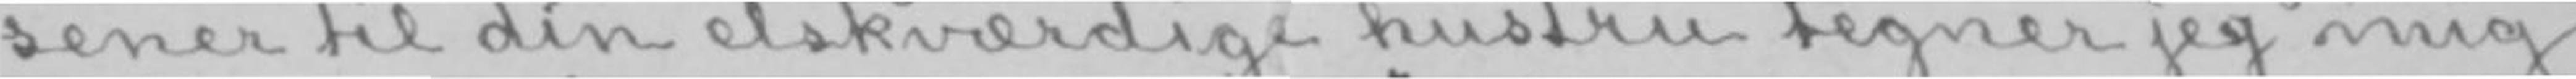sener til din elskværdige hustru tegner jeg mig
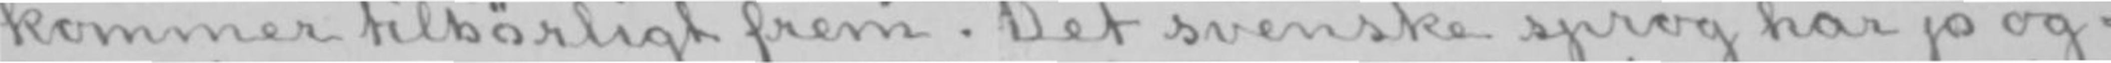kommer tilbørligt frem. Det svenske sprog har jo og-
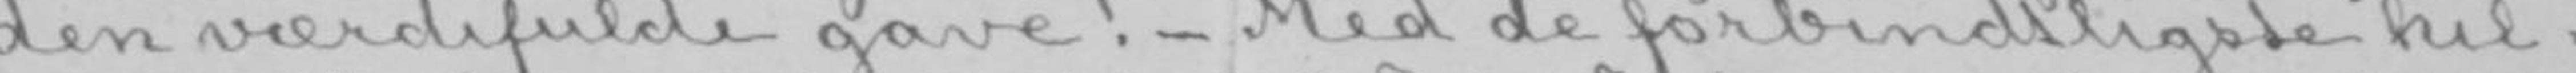den værdifulde gave!- Med de forbindtligste hil-
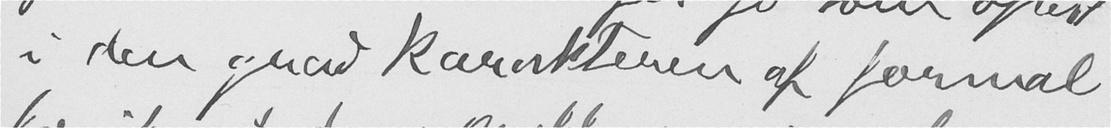i den grad karakteren af formal
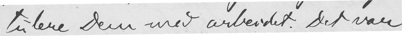tulere Dem med arbeidet. Det vare
